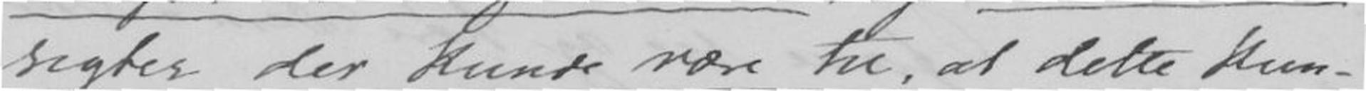sigter der kunde være til, at dette kun-
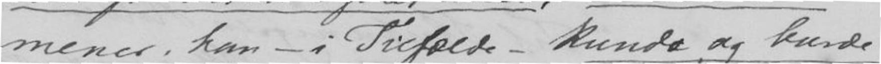mener, han - i Tilfælde - kunde og burde
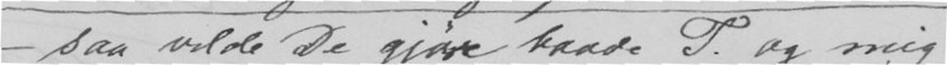- saa vilde De gjøre baade T. og mig
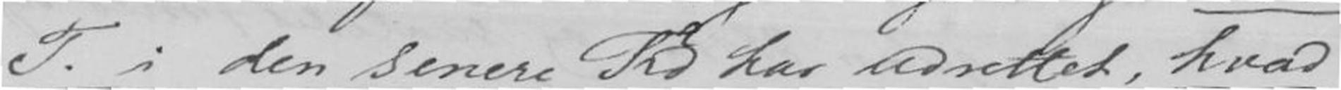T. i den senere Tid har udrettet, hvad
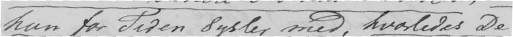han for Tiden sysler med, hvorledes De
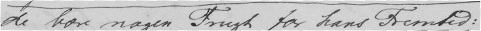de bære nogen Frugt for hans Fremtid:
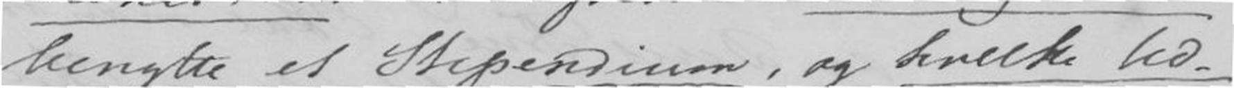benytte et Stipendium, og hvilke Ud-
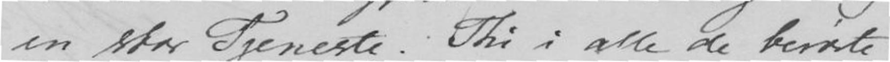en stor Tjeneste. Thi i alle de berørte
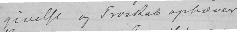givelse og Troskab ophæver
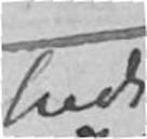hed
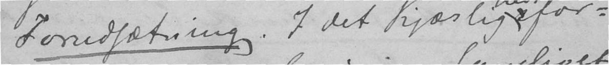Forudsætning. I det Kjærligsfor-
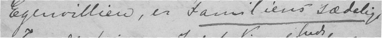Egenvillien, er Familiens sædelige
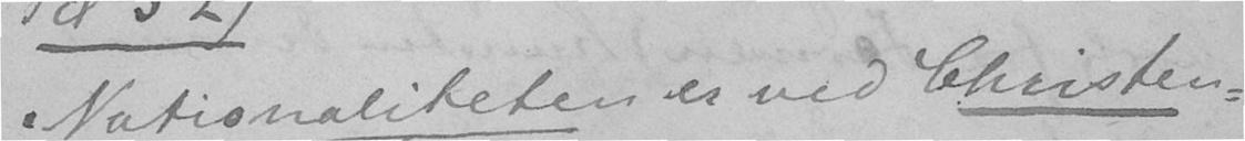Nationaliteten er ved Christen-
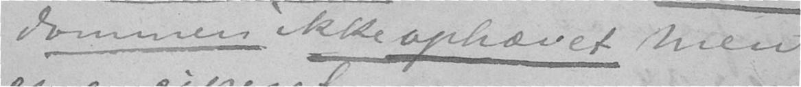dommen ikke ophævet, men
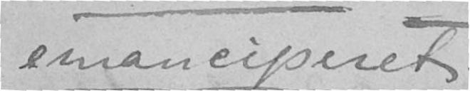emanciperet.
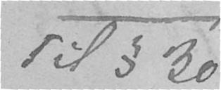Til § 30
